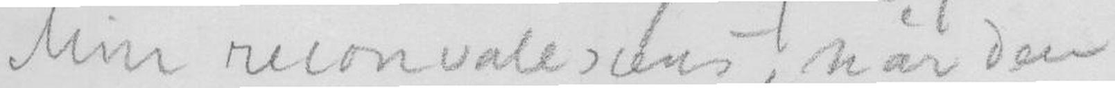Min reconvalescens, når den
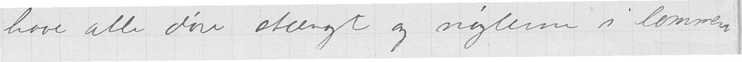have alle døre stengt og nøglerne i lommen
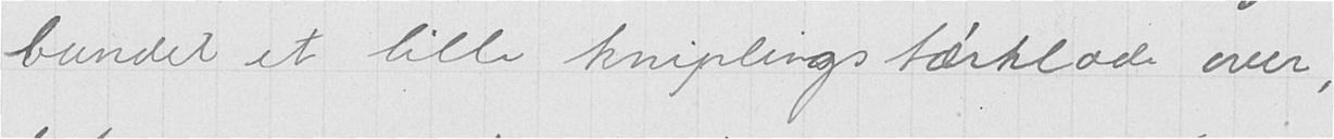bundet et lille kniplingstørklæde over,
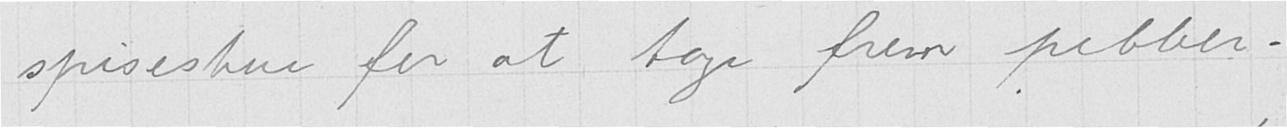spisestue for at tage frem pebber-
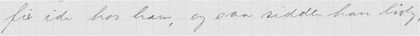fix ide hos ham, og saa sidder han livlig,
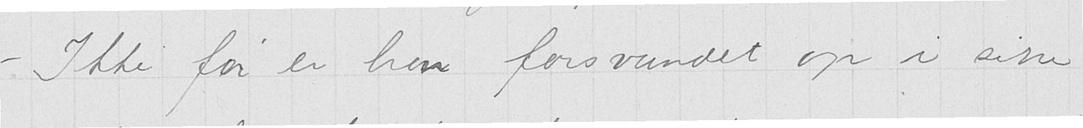- Ikke før er han forsvundet op i sine
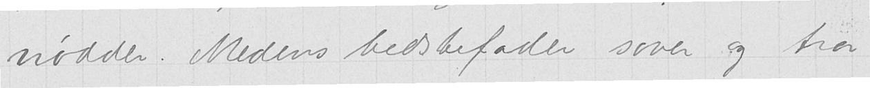nødder. Medens bedstefader sover og tror
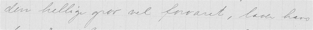den hellige grav vel forvaret, laver hans
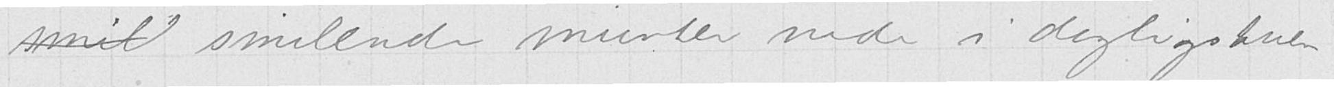smil smilende munter inde i dagligstuen
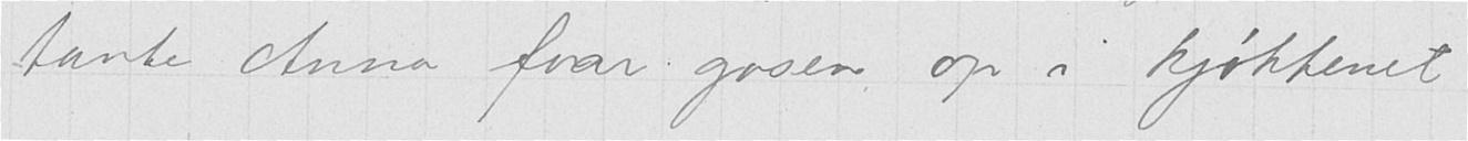tante Anna faar gasen op i kjøkkenet
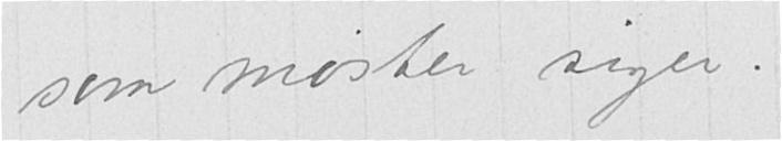som moster siger.
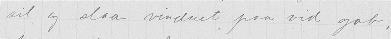sit og slaar vinduet paa vid gab,
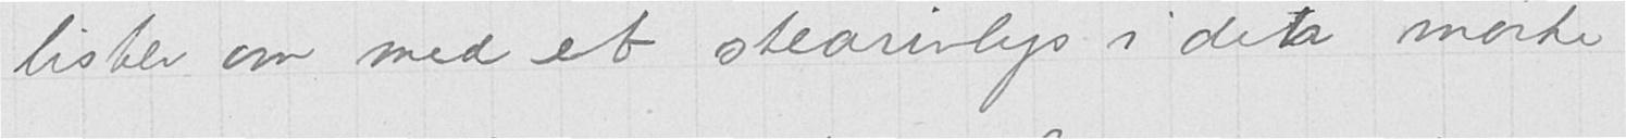lister om med et stearinlys i den mørke
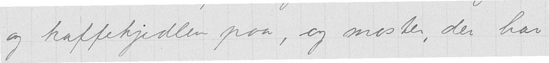og kaffekjedlen paa, og moster, der har
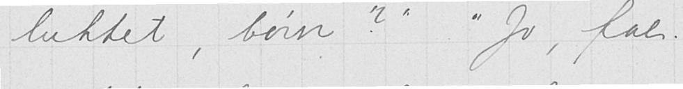lukket, børn?" "Jo, far."
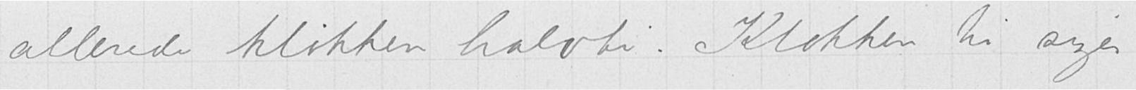allerede klokken halvti. Klokken ti siger
